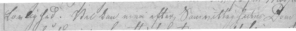Lovlighed. Vel kan man efter Samvittighedens Dom
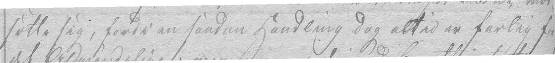sætte sig, fordi en saadan Handling dog altid er farlig for
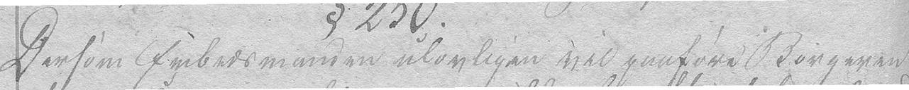Dersom Embedsmanden ulovligen vil paaføre Borgeren
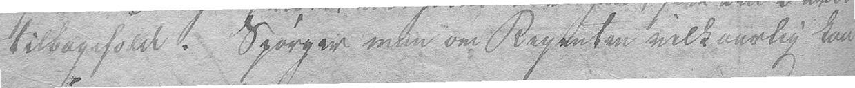tilbageholde. Spørger man om Regenten vilkaarlig kan
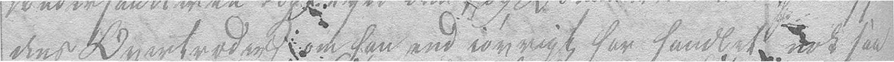dens Overtræder, om han end iøvrigt har handlet nok saa
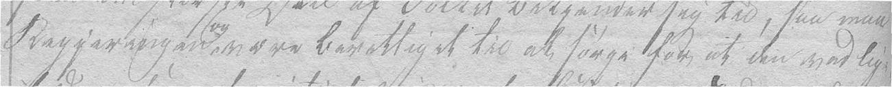Regjeringen være berettiget til at sørge for, at den vedlige-
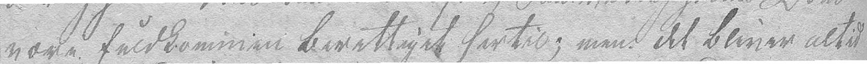være fuldkommen berettiget hertil; men det bliver altid
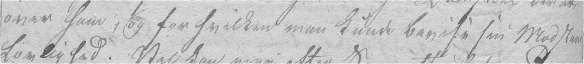over ham, og for hvilken man kunde bevise sin Modstands
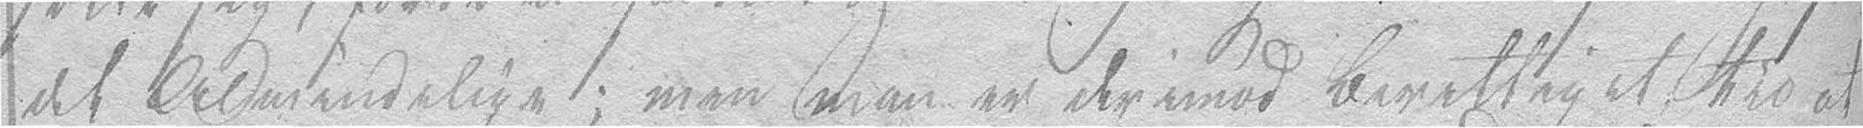det Almindelige; men man er derimod berettiget til at
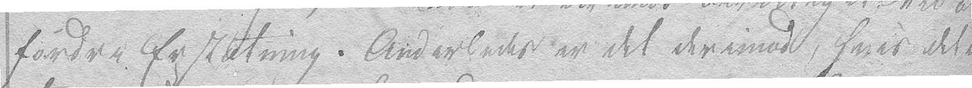fordre Erstatning. Anderledes er det derimod, hvis det er
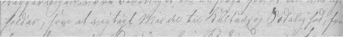holdes, som et vigtigt Middel til Kultur og Sædelighed, frem-
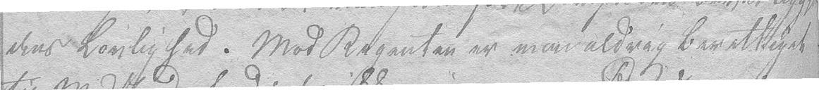dens Lovlighed. Mod Regenten er man aldrig berettiget
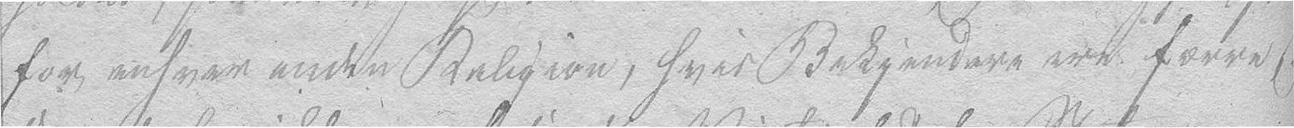for enhver anden Religion, hvis Bekiendere ere færre og
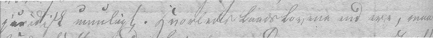juridisk umuligt. Hvorledes Landslovene end ere, maa
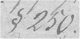§ 250.
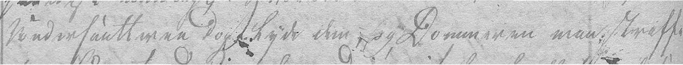Undersaatterne dog lyde dem, og Dommeren maa straffe
